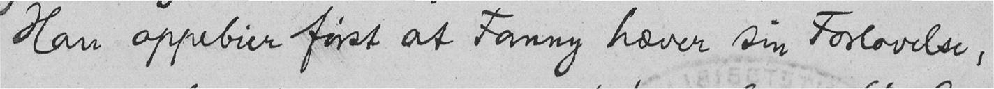Han oppebier først at Fanny hæver sin Forlovelse,
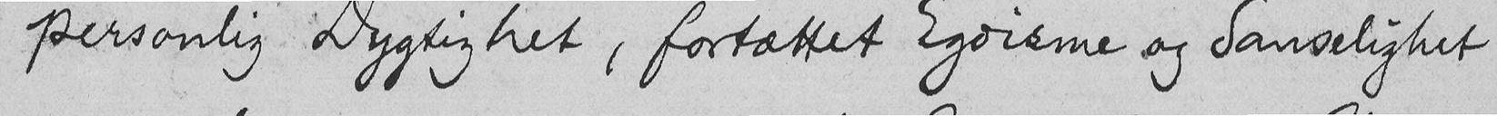personlig Dygtighet, fortættet Egoisme og Sanselighet
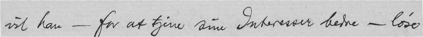vil han - for at tjene sine Interesser bedre - løse
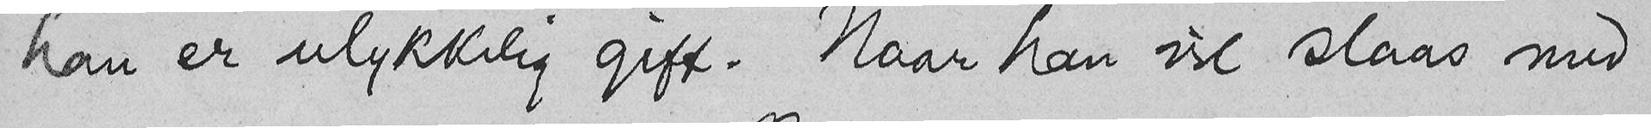han er ulykkelig gift. Naar han vil slaas med
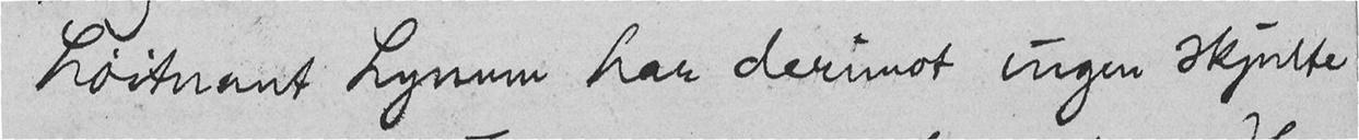Løitnant Lynum har derimot ingen skjulte
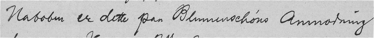Naboben er dette paa Blumenschøns Anmodning
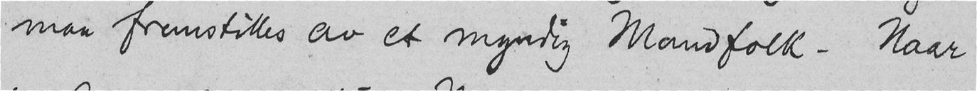maa fremstilles av et myndig Mandfolk. Naar
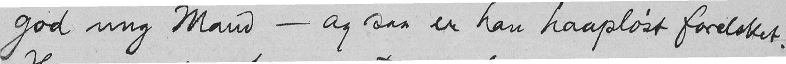god ung Mand - og saa er han haapløst forelsket.
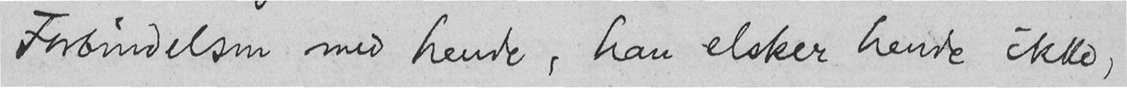Forbindelsen med hende, han elsker hende ikke,
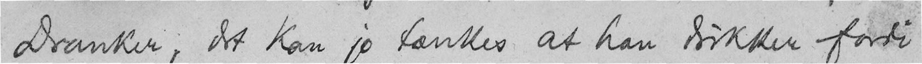Dranker, det kan jo tænkes at han drikker fordi
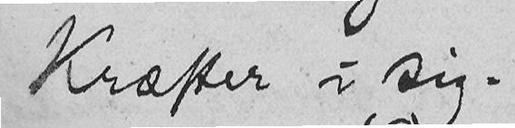Kræfter i sig.
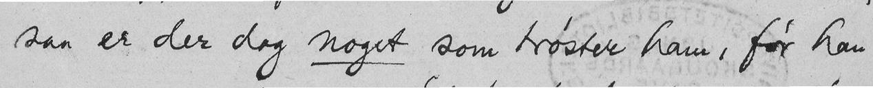saa er der dog noget som trøster ham, før han
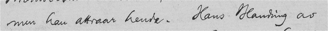men han attraar hende. Hans Blanding av
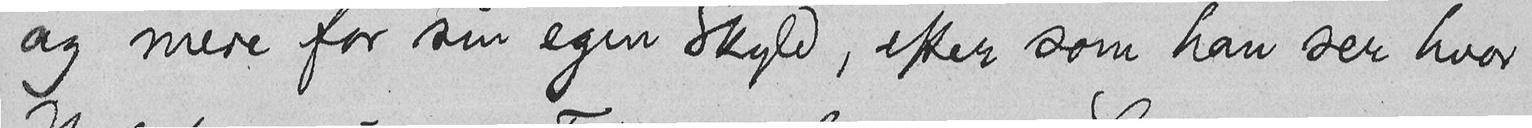og mere for sin egen Skyld, efter som han ser hvor
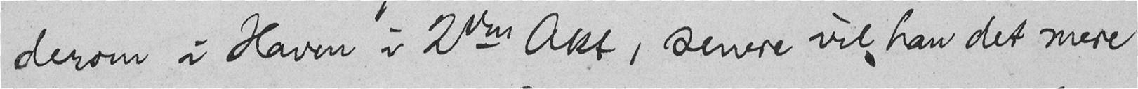derom i Haven i 2den Akt, senere vil han det mere
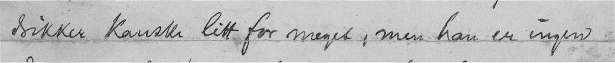drikker kanske litt for meget, men han er ingen
